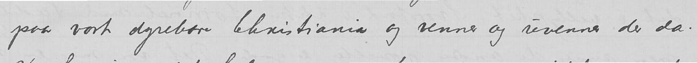paa vart dyrebare Christiania og venner og uvenner der da.
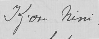Kjære Nini,
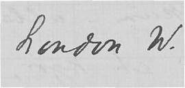London W.
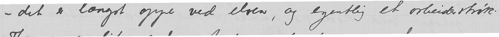– det er længst oppe ved elven, og egentlig et arbeiderstrøk.
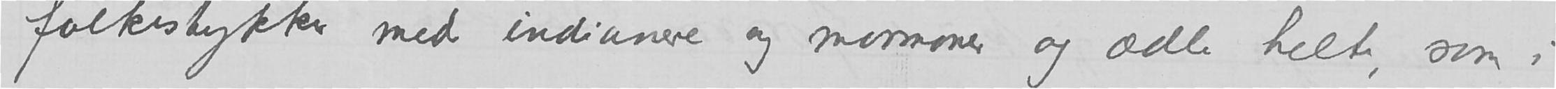folkestykker med indianere og mormoner og ædle helte, som i
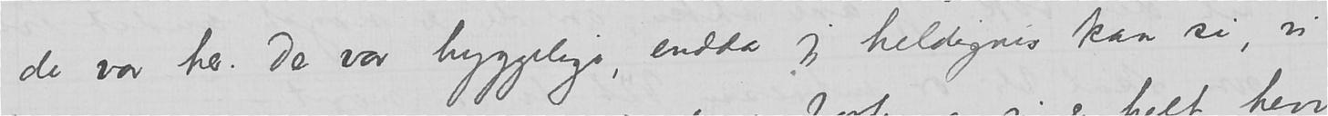de var her. De var hyggelige, endda jeg heldigvis kan si, vi
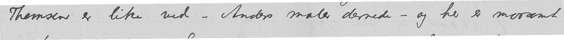Themsen er like ved – Anders maler dernede – og her er morsomt
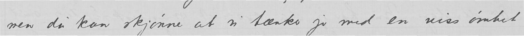men du kan skjønne at vi tænker jo med en viss ømhet
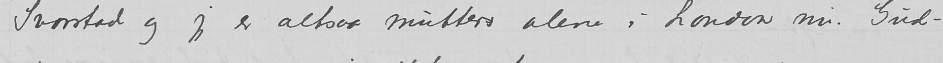Svarstad og jeg er altsaa mutters alene i London nu. Gud-
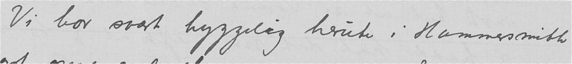Vi bor svært hyggelig herute i Hammersmith
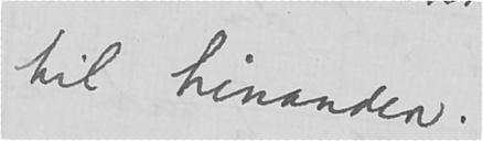til hinanden
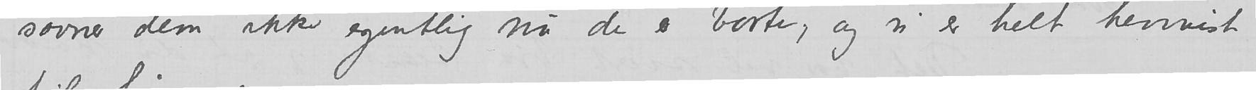savner dem ikke egentlig nu de er borte, og vi er helt henvist
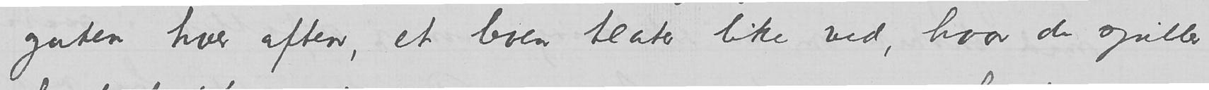gaten hver aften, et leven teater like ved, hvor de spiller
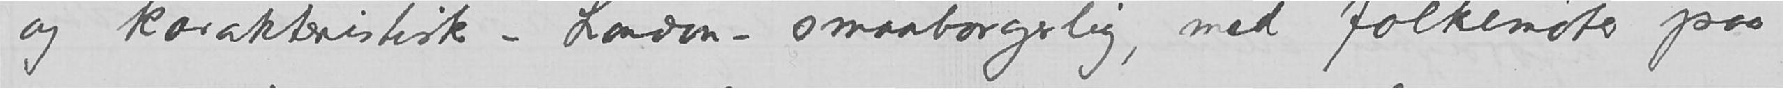og karakteristisk – London- smaaborgerlig, med folkemøter paa
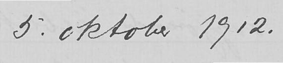5. oktober 1912.
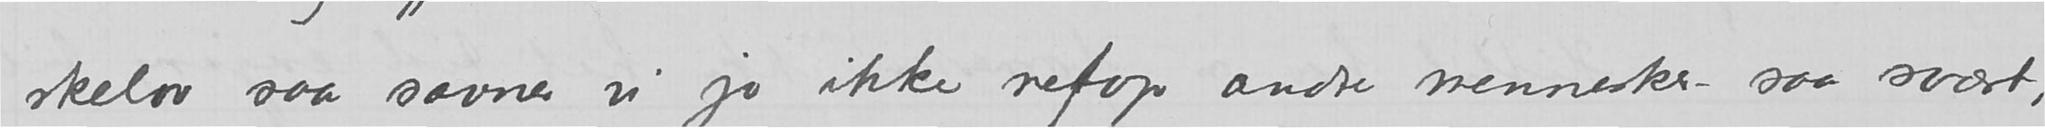skelov saa savner vi jo ikke netop andre mennesker saa svært,
